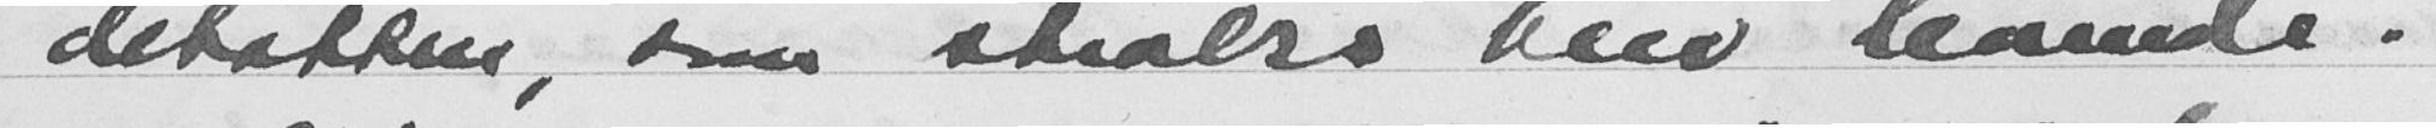debatten, som straks blev levende!
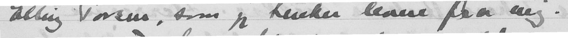Elling Torsen, som jeg tenker levere fra mig.
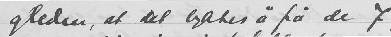gleden, at det lykkes å få de 7
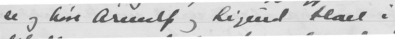se og høre Arnulf og Sigurd Hoel i
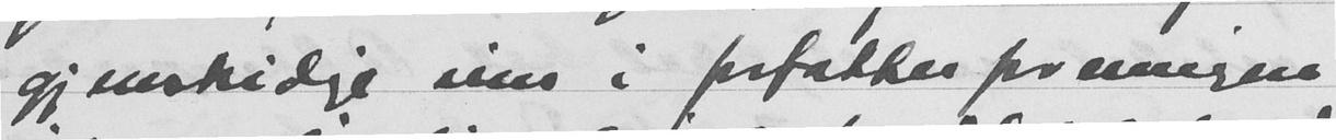gjenstridige inn i forfatterforeningen
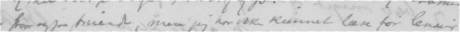stoor ogsaa smaadt, men jeg har ikke kunnet læse før Censur
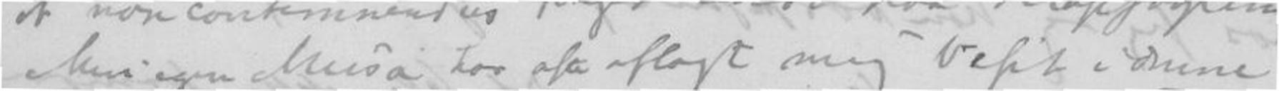Min egen Musa har ofte aflagt mig Visit i denne
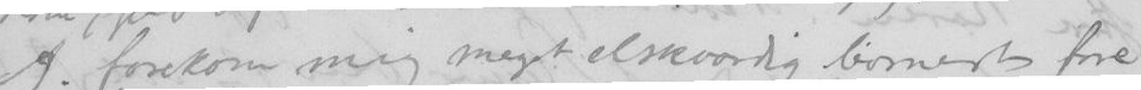A. forekom mig meget elskværdig, bomert føre-
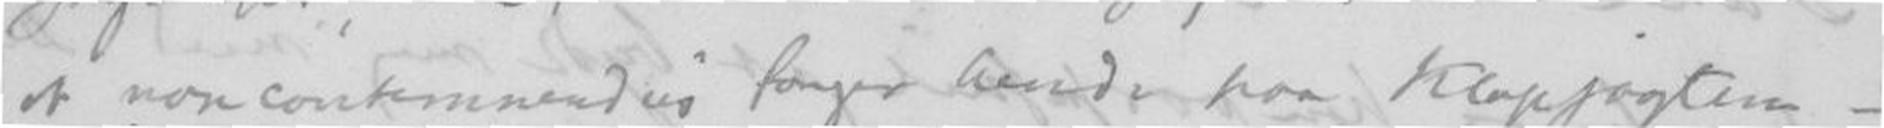et noncontemnendus fanger hende paa Klapjagten -
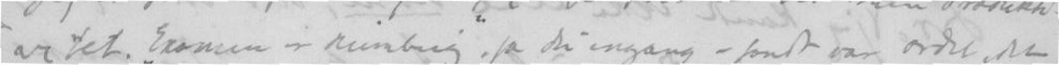vitet. "Examen er humbug", sa du engang - sandt var ordet det
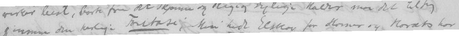gjennem den herlige Fantasi; Men lidt Elskov for Homer og Harats har
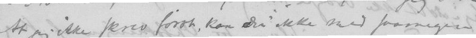At jeg ikke skrev først, kan du ikke med formegen
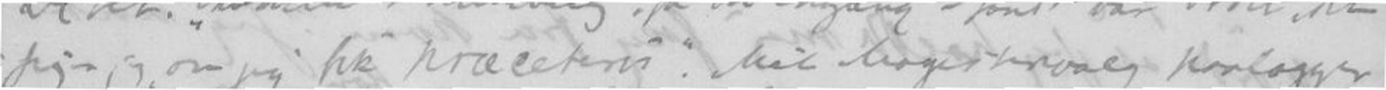siger jeg om jeg fik præcetens". Mit Magistervalg paalægger
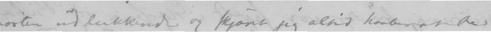næsten udelukkende og skjønt jeg altid haaber at de
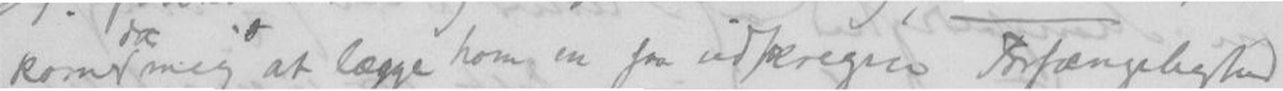kom det mig at lægge ham en saa udpregen. Forfængelighed
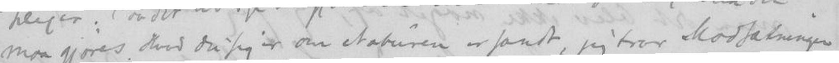maa gjøres. Hvad du siger om Naturen er Sandt, jeg tror Modsætningen
